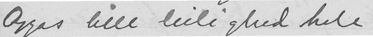Aggas lille leilighed hele
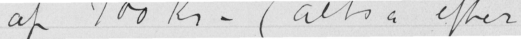af 700 Kr – (altså efter
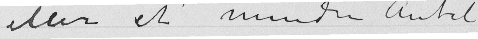eller et mindre Antal
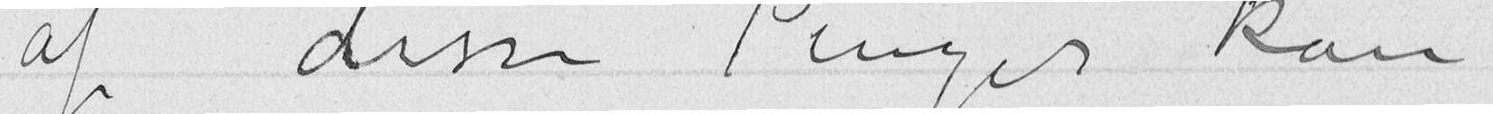af disse Penger kan
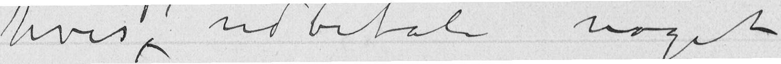hvis jeg udbetaler noget
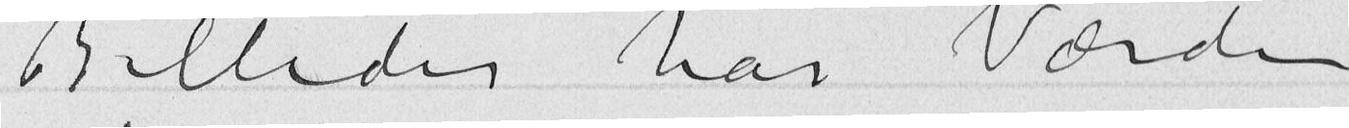Billeder har Værdi
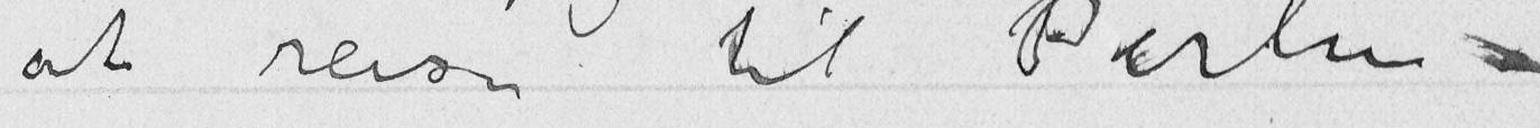at reise til Berlin –
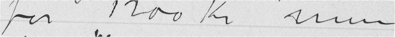for 1200 Kr men
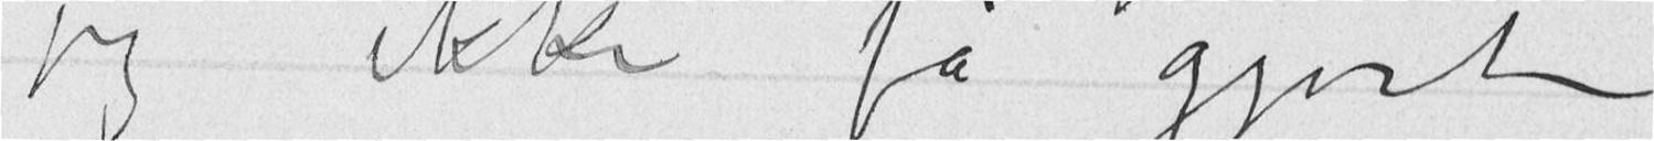jeg ikke få gjort
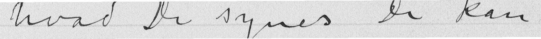hvad De synes De kan
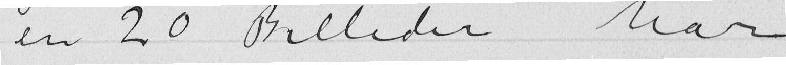en 20 Billeder har
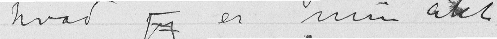hvad jeg er min akt
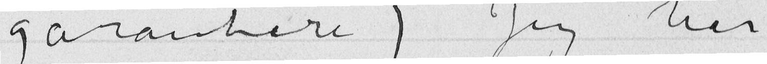garantere) Jeg har
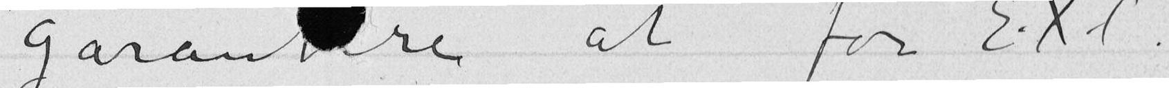garantere at for E.X.l
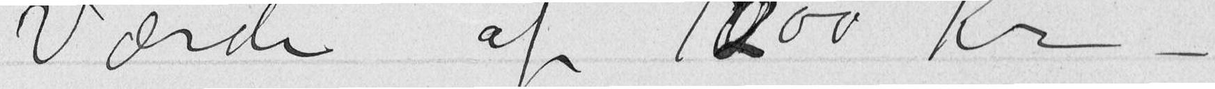Værdi af 1200 Kr –
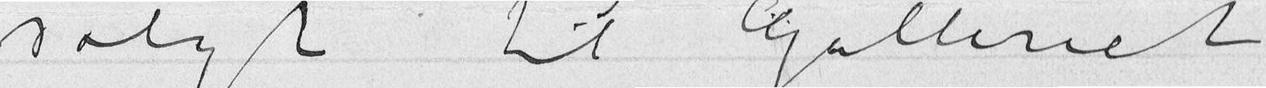solgt til Galleriet
